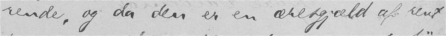rende, og da den er en æresgjæld af rent
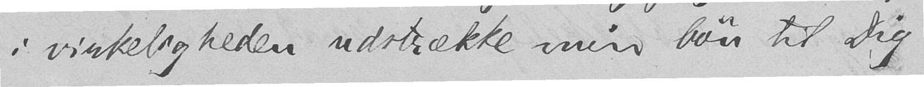i virkeligheden udstrække min bøn til Dig
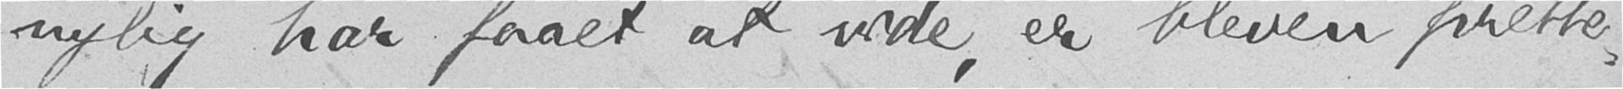nylig har faaet at vide, er bleven presse-
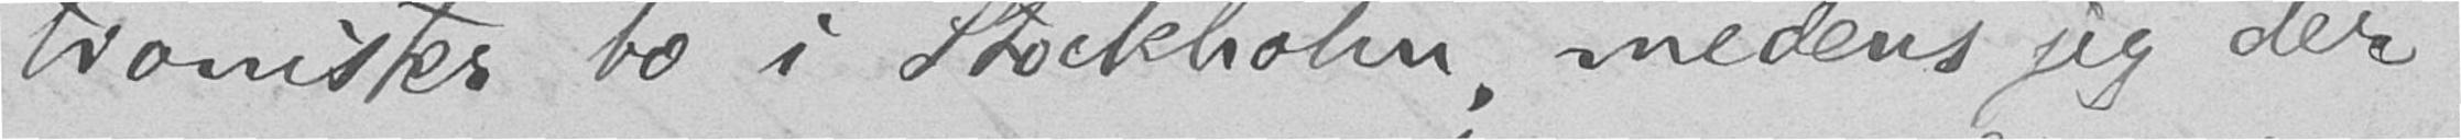tionister bo i Stockholm, medens jeg der
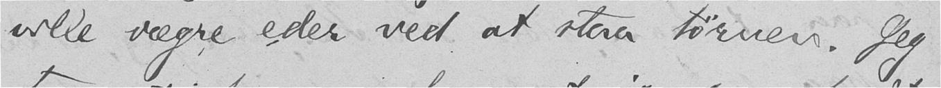ville vægre eder ved at staa tørnen. Jeg
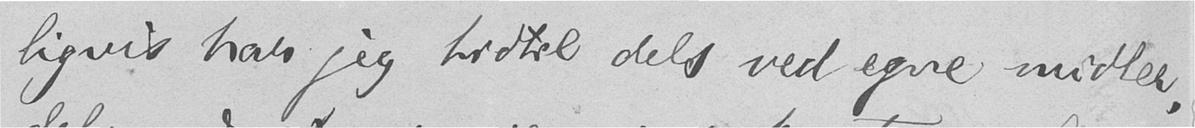ligvis har jeg hidtil dels ved egne midler,
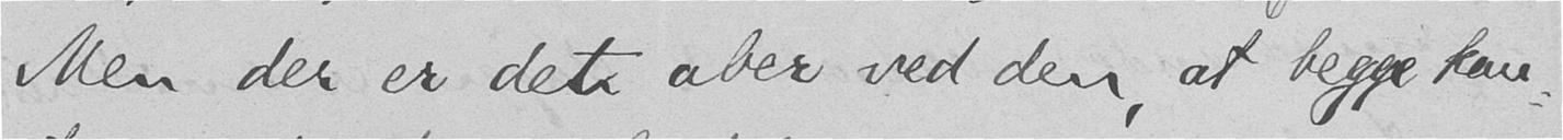Men der er det aber ved den, at begge kau-
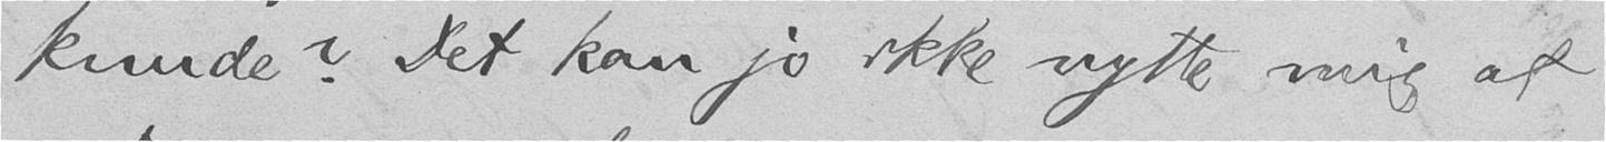knude? Det kan jo ikke nytte mig at
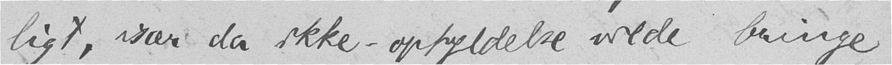ligt, især da ikke-opfyldelse vilde bringe
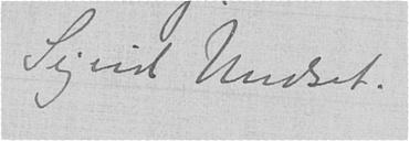Sigrid Undset.
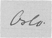Oslo.
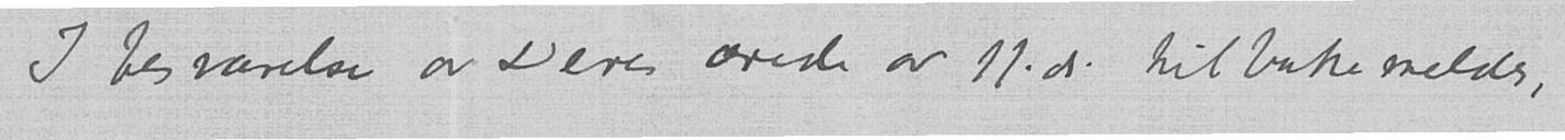I besvarelse av Deres ærede av 11. ds. tilbakemeldes,
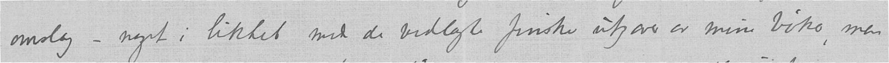omslag – noget i liktet med de vedlagte finske utgaver av mine bøker, men
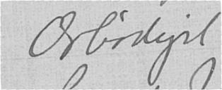Ærbødigst
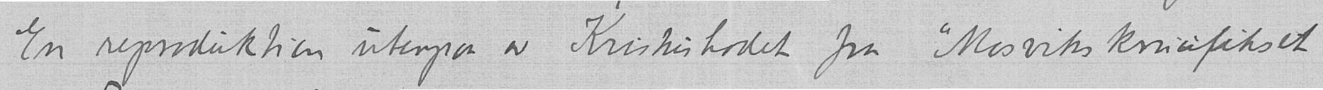En reproduktien utenpaa av Kristushodet fra "Mosvikskrusifikset"
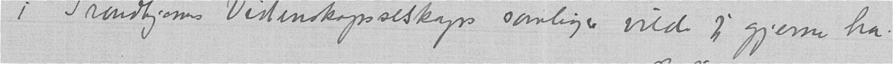i Trondhjems Videnskapsselskaps samlinger vilde jeg gjerne ha.
